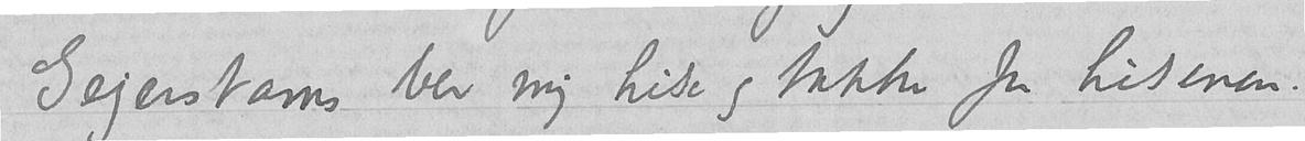Gejerstams ber mig hilse og takke for hilsenen.
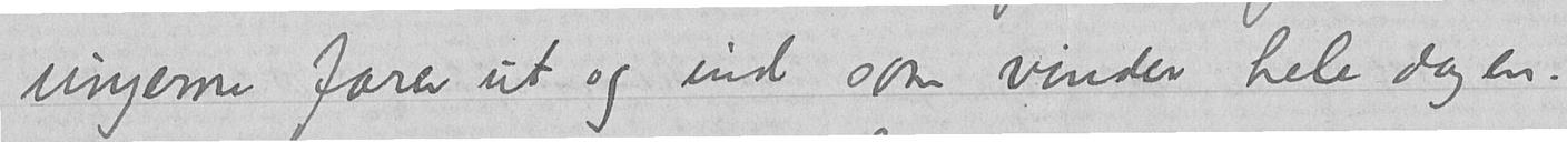ungerne farer ut og ind som vinder hele dagen.
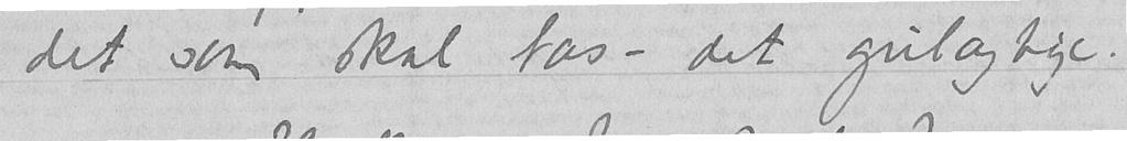det som skal tas – det gulagtige.
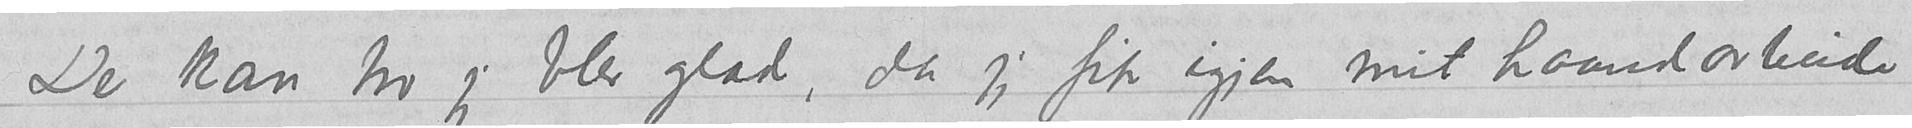De kan tro jeg blev glad, da jeg fik igjen mit haandarbeide
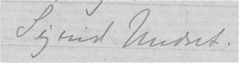Sigrid Undset.
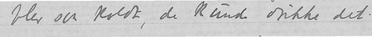blev saa koldt, de kunde drikke det.
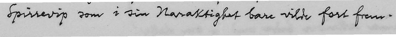Spirrevip som i sin Naraktighet bare vilde fort frem.
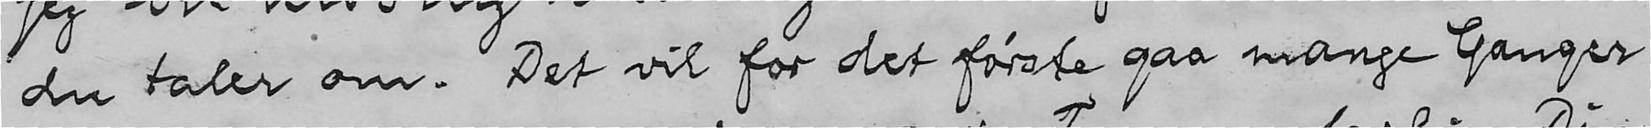du taler om. Det vil for det første gaa mange Ganger
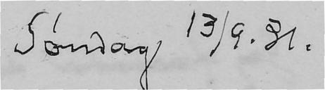Søndag 13/9. 31.
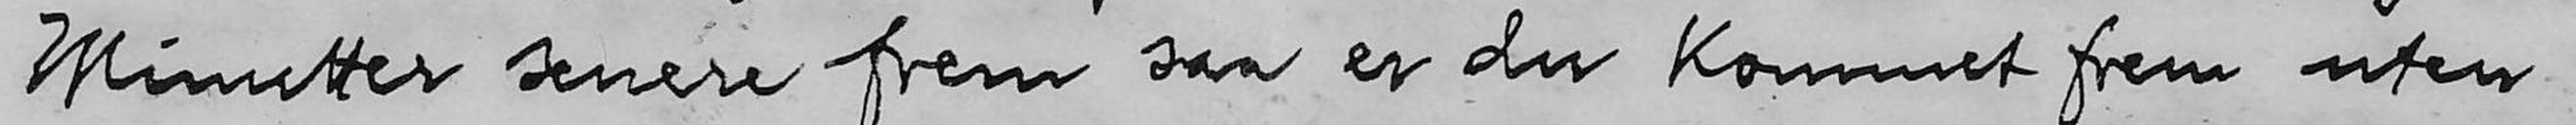Minutter senere frem saa er du kommet frem uten
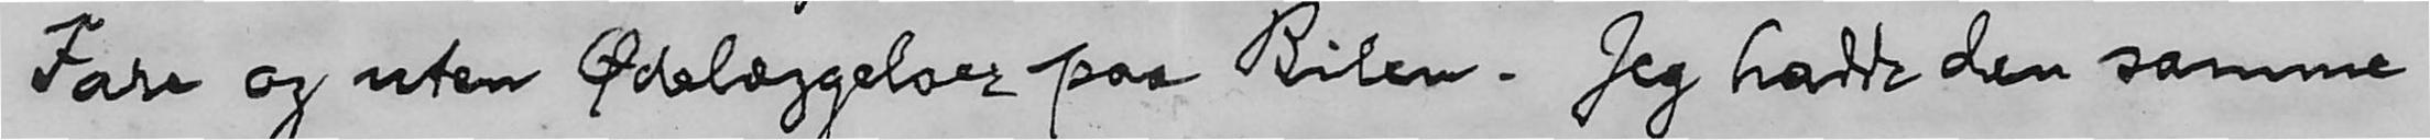Fare og uten Ødelæggelser paa Bilen. Jeg hadde den samme
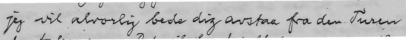jeg vil alvorlig bede dig avstaa fra den Turen
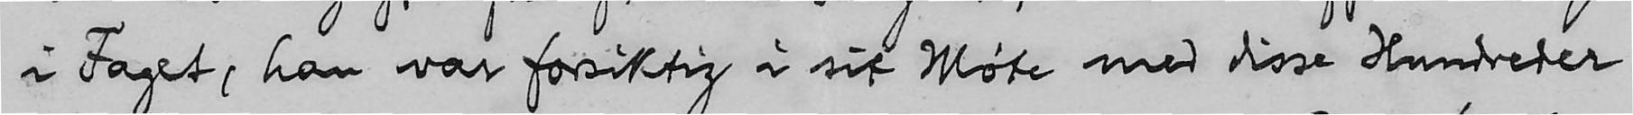i Faget, han var forsiktig i sit Møte med disse Hundreder
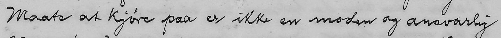Maate at kjøre paa er ikke en moden og ansvarlig
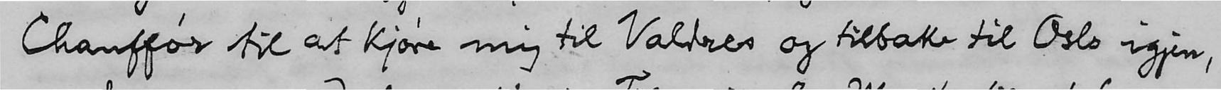Chauffør til at kjøre mig til Valdres og tilbake til Oslo igjen,
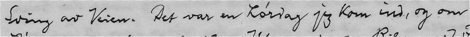Sving av Veien. Det var en Lørdag jeg kom ind, og om
